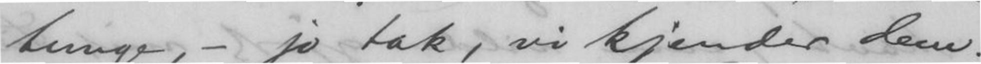tunge, - jo tak, vi kjender dem.
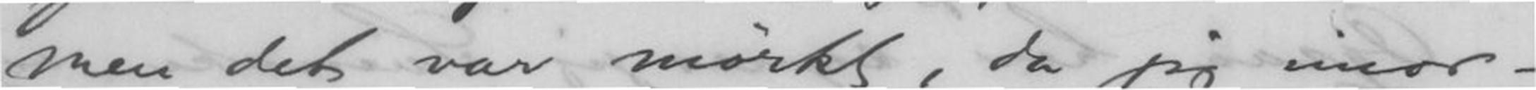men det var mørkt, da jeg imor-
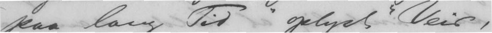paa lang Tid oplyst Veir,
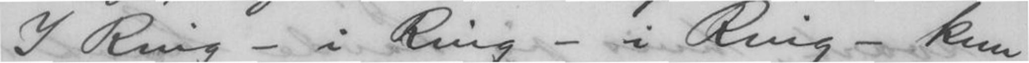I Ring - i Ring - i Ring - kun
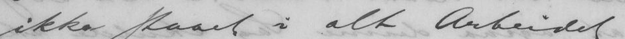ikke staaet i alt Arbeidet.
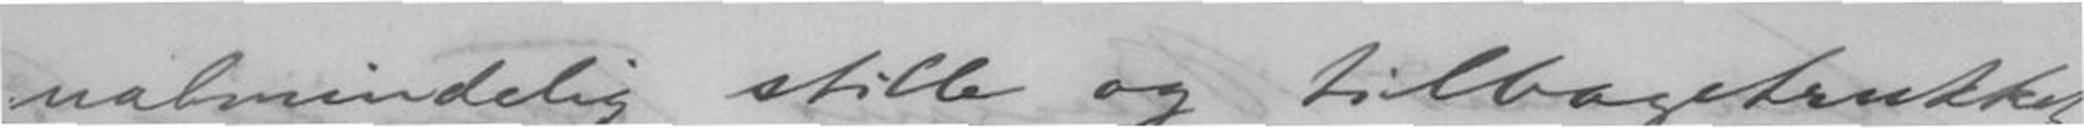ualmindelig stille og tilbagetrukket,
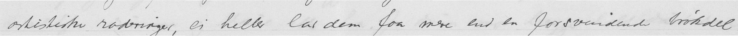artistiske raderinger, ei heller lar dem faa mere end en forsvindende brøkdel
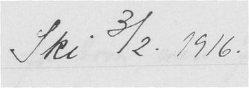Ski 3/2 1916.
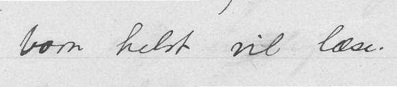barn helst vil læse.
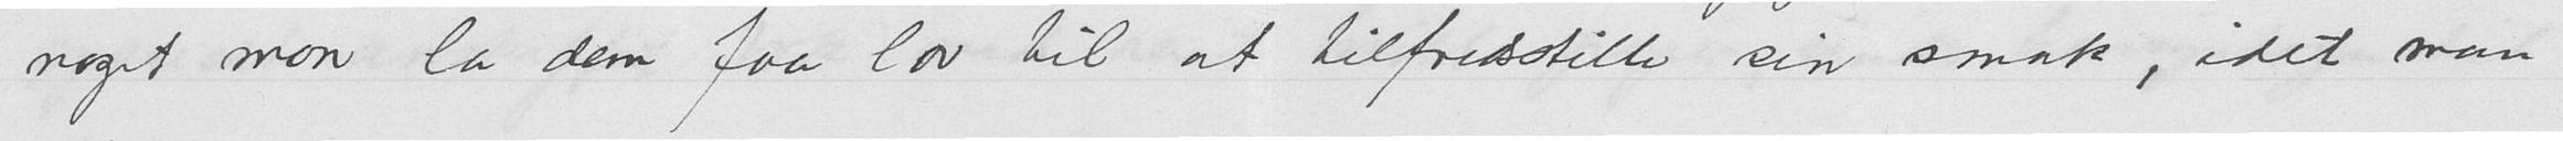noget mon la dem faa lov til at tilfredsstille sin smak, idet man
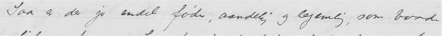Saa er der jo endel føde, aandelig og legemlig, som baade
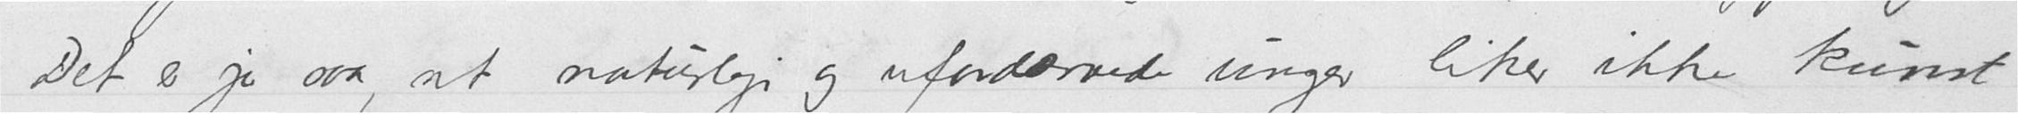Det er jo saa, at naturlige og ufordærvede unger liker ikke kunst
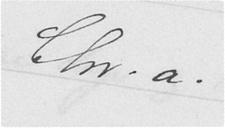Chr.a.
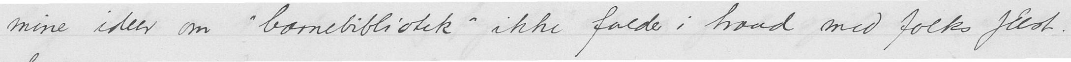mine ideer om "barnebibliotek" ikke falder i traad med folks flest.
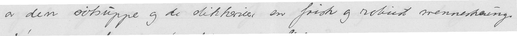av den søtsuppe og de slikkerier en frisk og robust menneskeunge
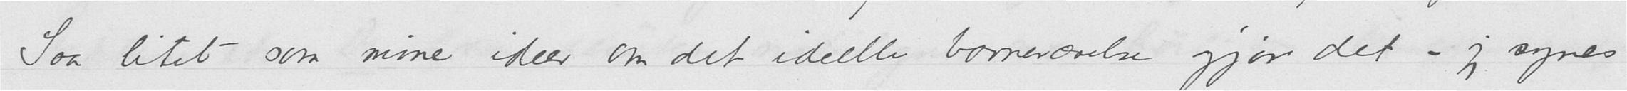Saa litet som mine ideer om det ideelle børneværelse gjør det - jeg synes
